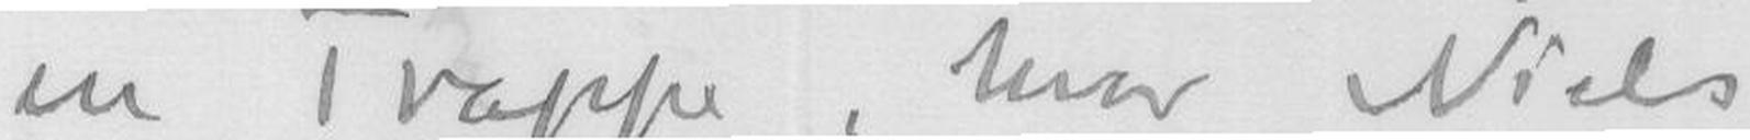en Trappe, hvor Niels
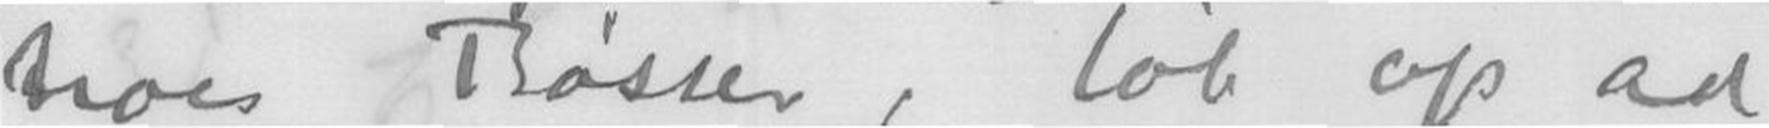troes Bøsser, løb op ad
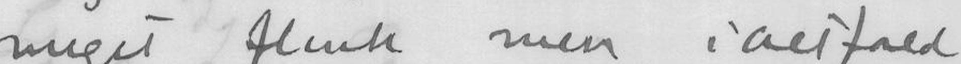meget flink men ialtfald
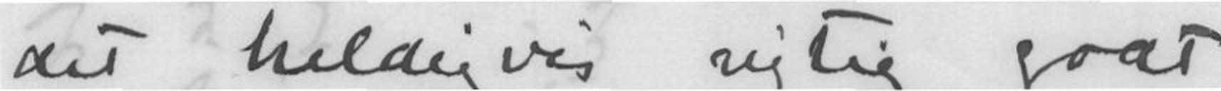det heldigvis rigtig godt
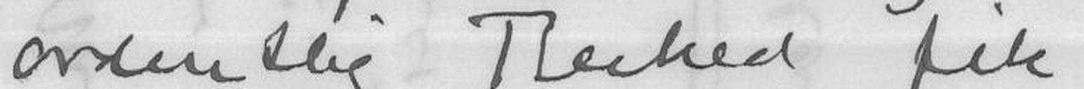ordentlig Besked fik.
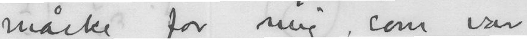måske for mig, som var
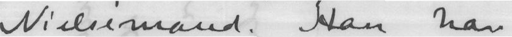Nielsemand. Han har
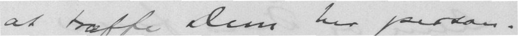at træffe Dem her person-
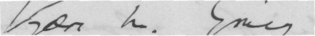Kjære hr. Grieg!
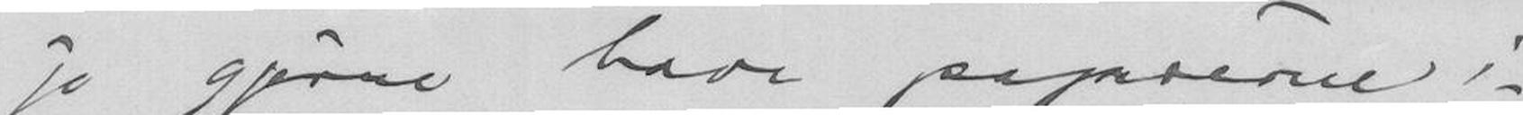jo gjerne have papirerne i-
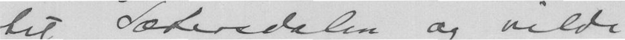til Sætersdalen og vilde
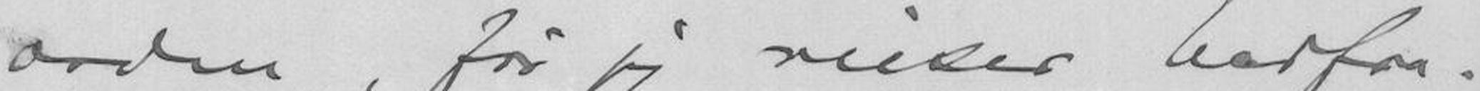orden, før jeg reiser herfra.
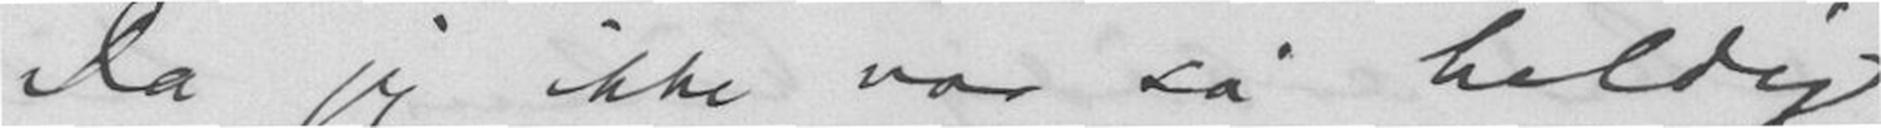Da jeg ikke var så heldig
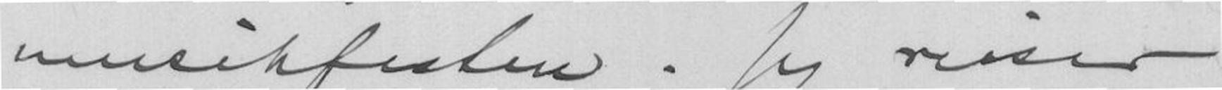musikfesten. Jeg reiser
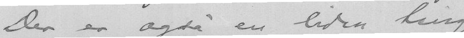Der er også en liden ting
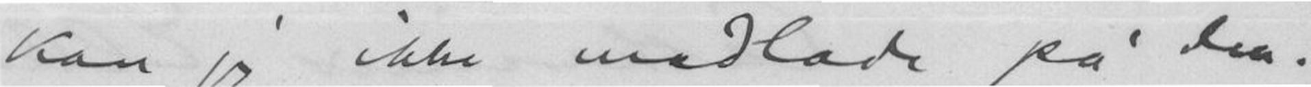kan jeg ikke medlade på den-
